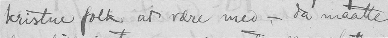kristne folk at være med, - da maatte
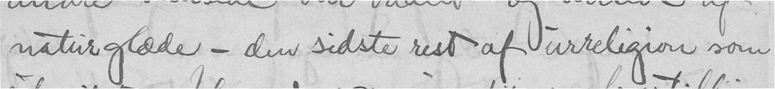naturglæde - den sidste rest af urreligion som
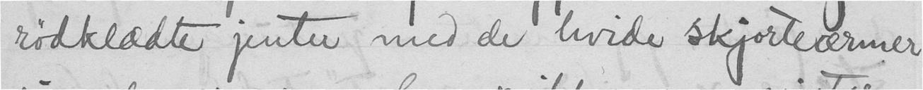rødklædte jenter med de hvide skjorteærmer
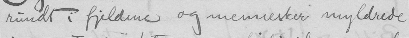rundt i fjeldene og mennesker myldrede
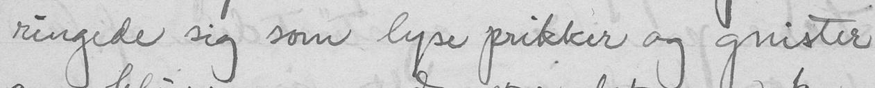ringede sig som lyse prikker og gnister
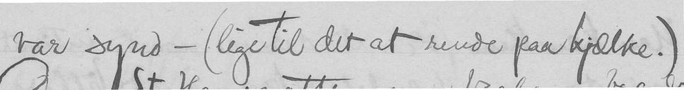var synd - (lige til det at rende paa kjælke.)
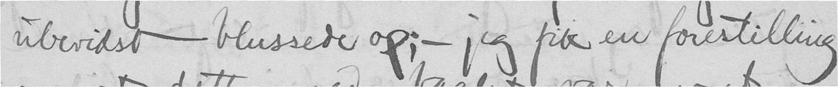ubevidst blessede op; - jeg fik en forestilling
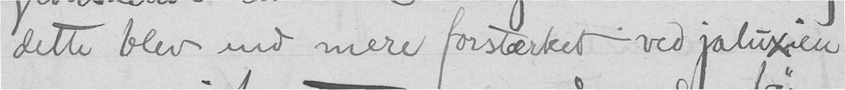dette blev end mere forstærket ved jaluxien
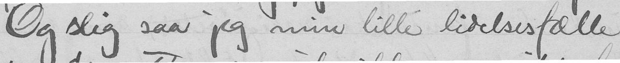Og slig saa jeg min lille lidelsesfælle
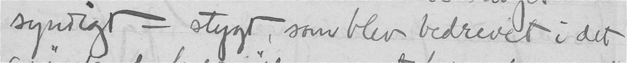syndigt - stygt, som blev bedrevet i det
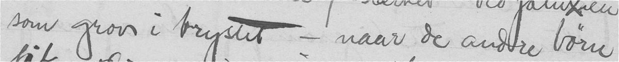som grovs i brystet - naar de andrre børn
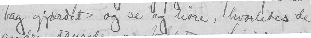bag gjærdet og se og høre, hvorledes de
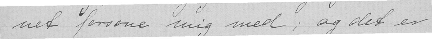net forsone mig med; og det er
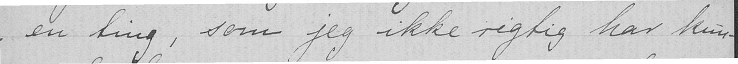en ting, som jeg ikke rigtig har kun-
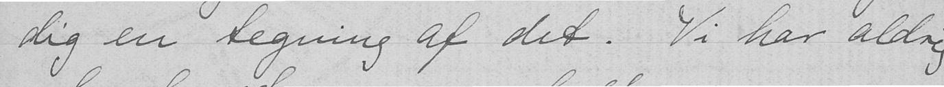dig en tegning af det. Vi har aldrig
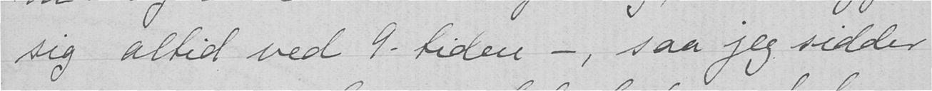sig altid ved 9- tiden -, saa jeg sidder
